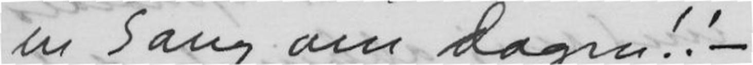en Gang om Dagen!!-
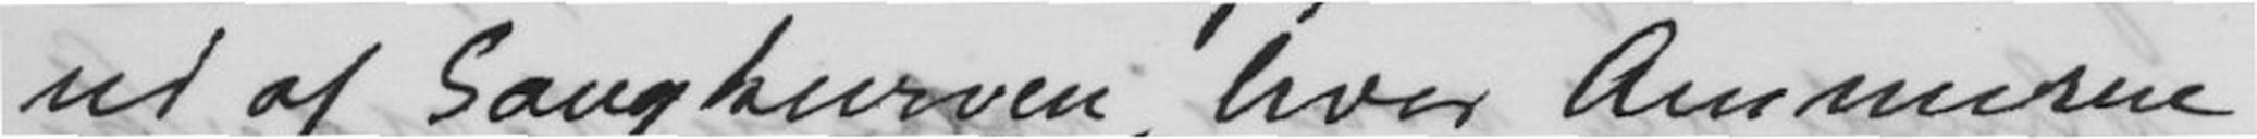ud af Sangkurven, hvor Ammerne
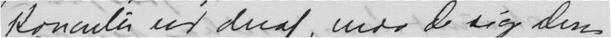Koncerter ud deraf, maa De sige Deres
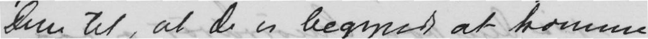Dem til, at De er begyndt at komme
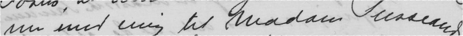me med mig til Madam Tusseand
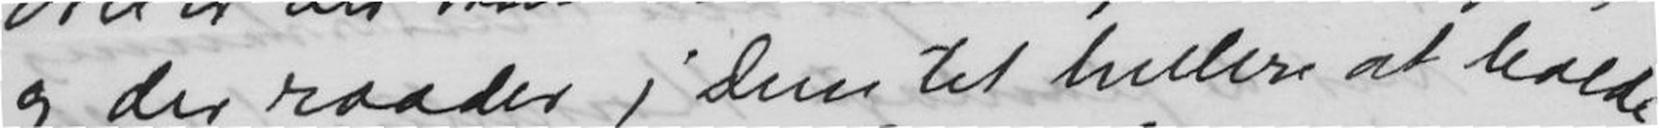og der raader j Dem til hellere at holde,
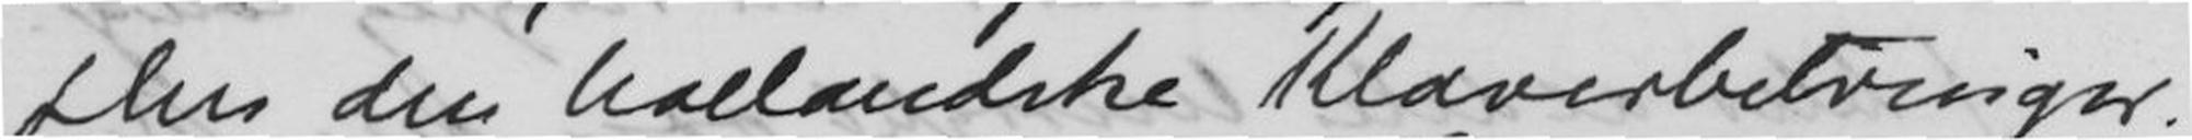pluss den hollandske Klaverbetvinger.
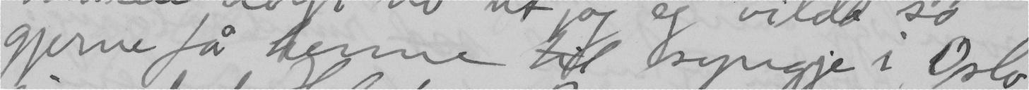gjerne få henne til syngje i Oslo
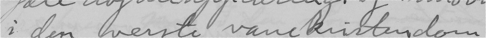i den verste vanekristendom,
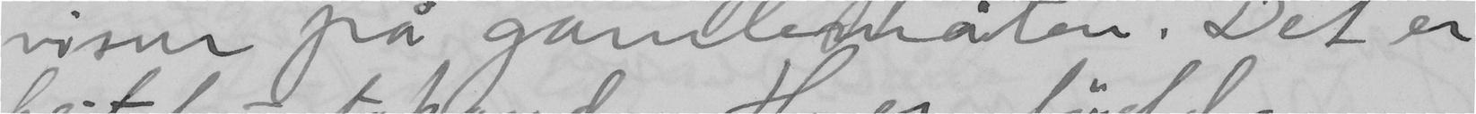visu på gamlemåten. Det er
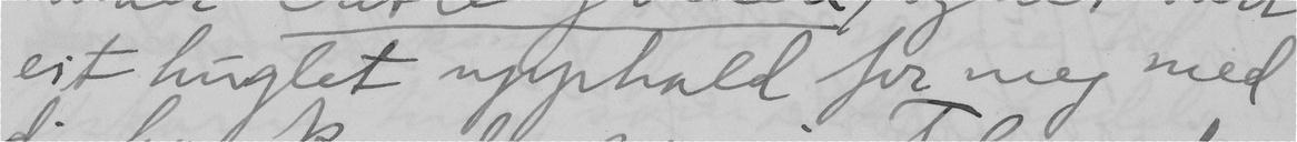eit huglet upphald for meg med
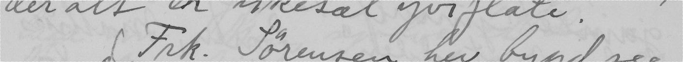ein  moderne liten heim
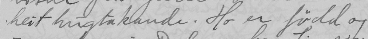heit hugtakande. Ho er född og
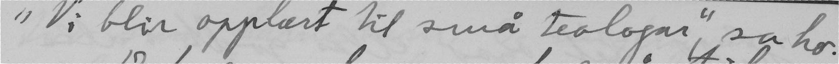"Vi blir opplært til små teologar", sa ho.
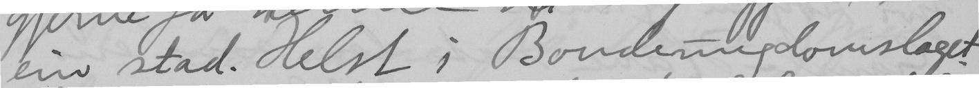ein stad. Helst i Bondeungdomslaget
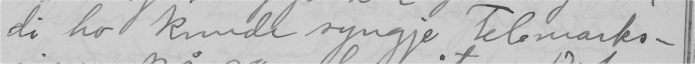di ho kunde syngje Telemarks-
